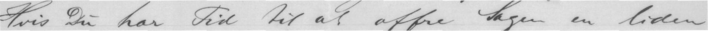Hvis Du har Tid til at offre Sagen en liden
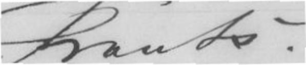Frants.
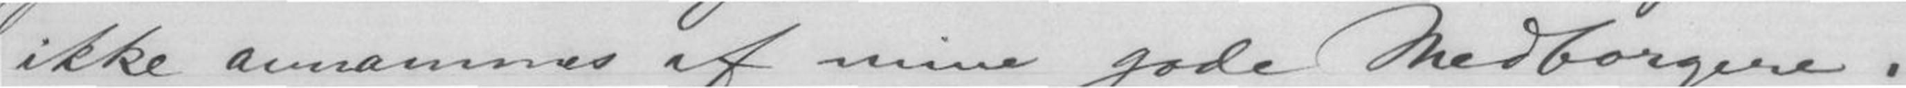ikke annammes af mine gode Medborgere,
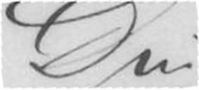Din
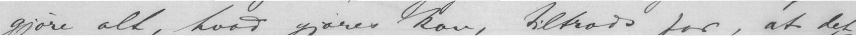gjøre alt, hvad gjøres kan, tiltrods for, at det
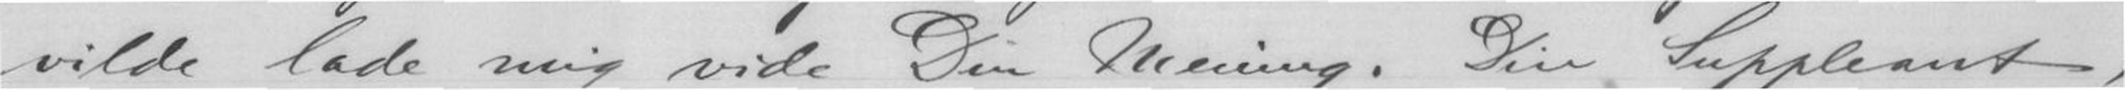vilde lade mig vide Din Mening. Din Suppleant,
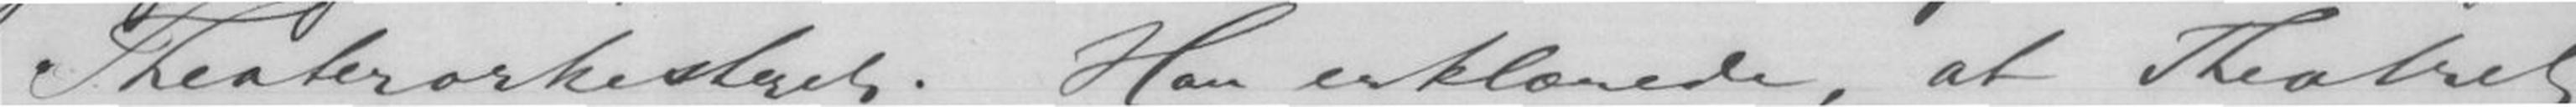Theaterorkesteret. Han erklærede, at Theatret
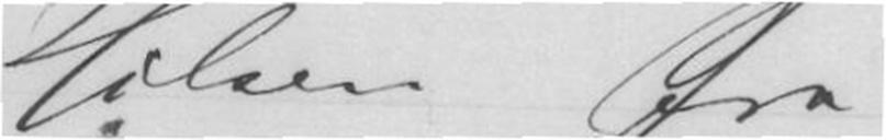Hilsen fra
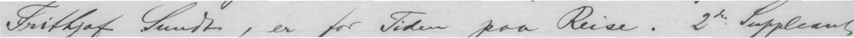Frithjof Sundt, er for Tiden paa Reise. 2den Suppleant
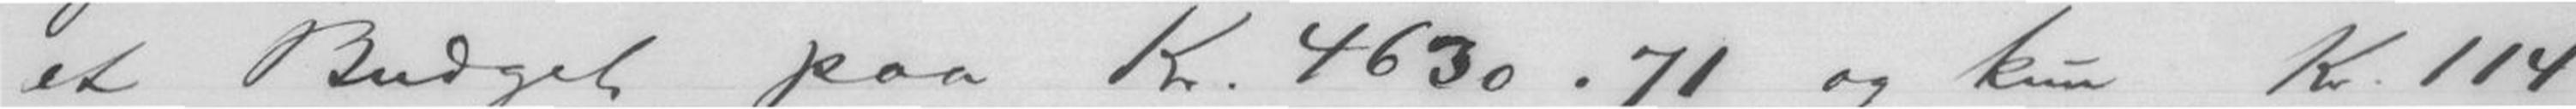et Budget paa Kr. 4630.71 og kun Kr. 114
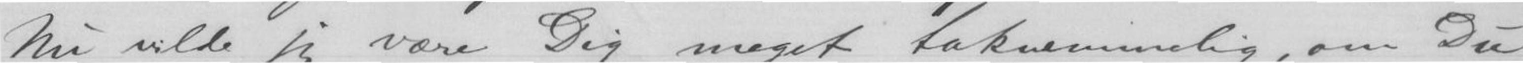Nu vilde jeg være Dig meget taknemmelig, om Du
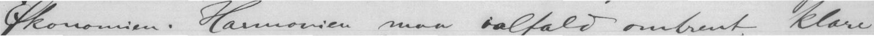Økonomien. Harmonien maa ialfald omtrent klare
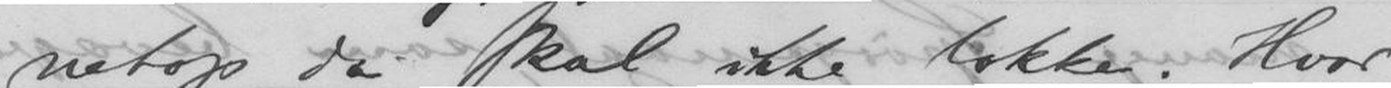netop da skal ikke lokke. Hvor
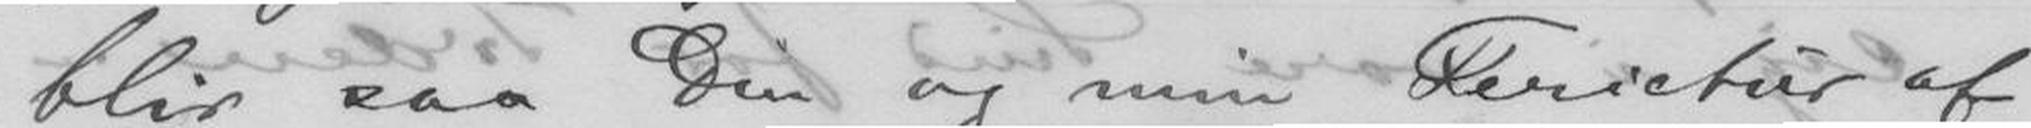blir saa Din og min Ferietur af
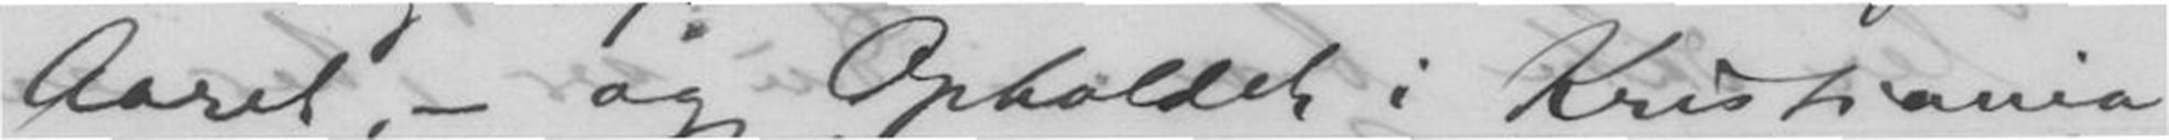Aaret, - og Opholdet i Kristiania
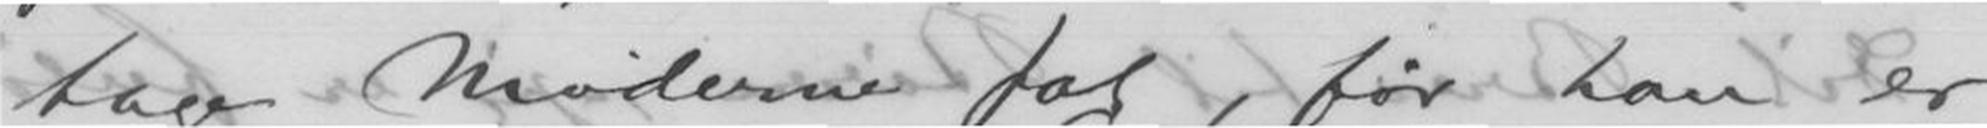tage Møderne fat, før han er
